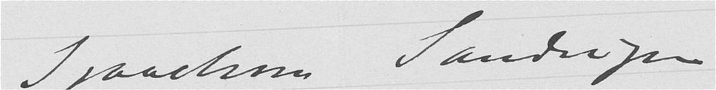Isaachsen Sandvigen
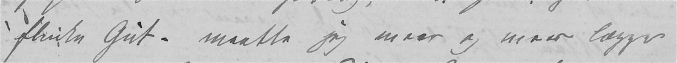flinke Gut – maatte jeg meer og meer lægge
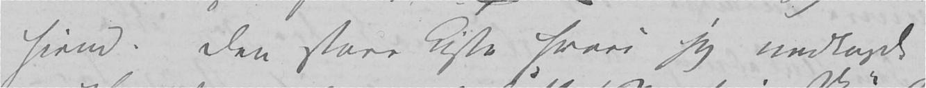hjem. Den store Kiste hvori jeg nedlagde
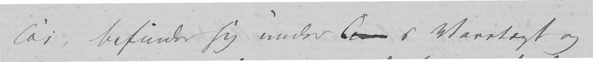Tøi, befinder sig under Cicis Varetægt og
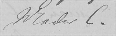Moder C.
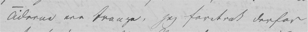Tiderne ere trange, jeg foretrak derfor
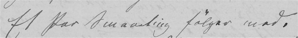Et Par Smaaeting følger med,
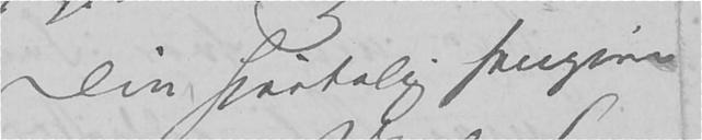Din hjertelig hengivne
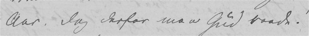Aar. Dog derfor maa Gud raade!
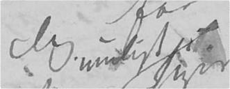Dig muligt
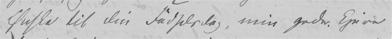Ønske til Din Fødselsdag, min gode, kjere
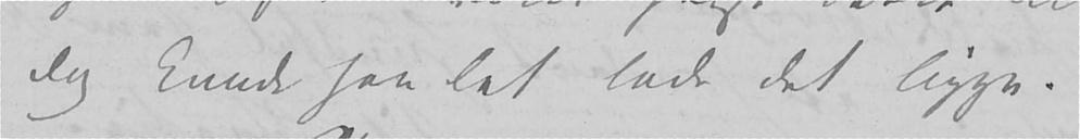dog kunde saa let lade det ligge.
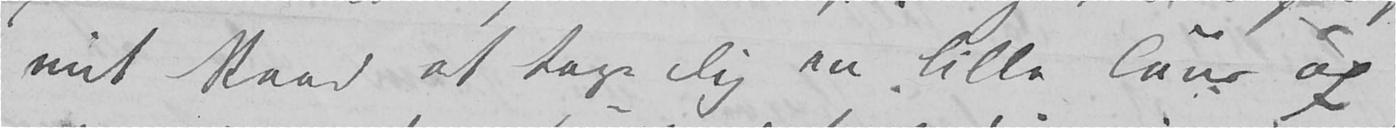mit Raad at tage Dig en lille Tour op
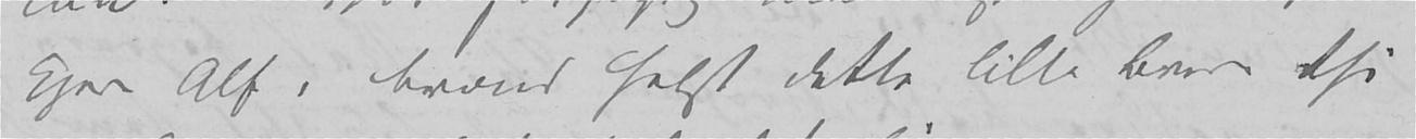kjere Alf, brænd helst dette lille Brev thi
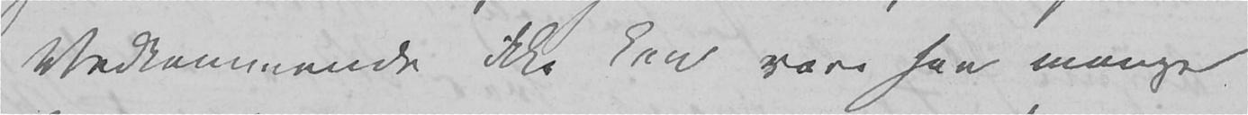Vedkommende ikke kan være saa mange
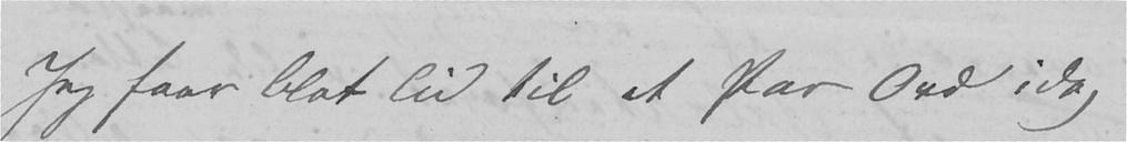Jeg faar blot Tid til et Par Ord idag
